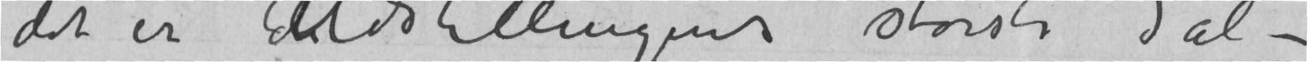Det er Udstillingens største Sal –
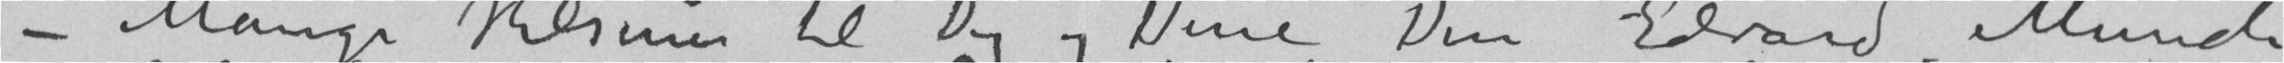– Mange Hilsener til Dig og Dine Din Edvard Munch
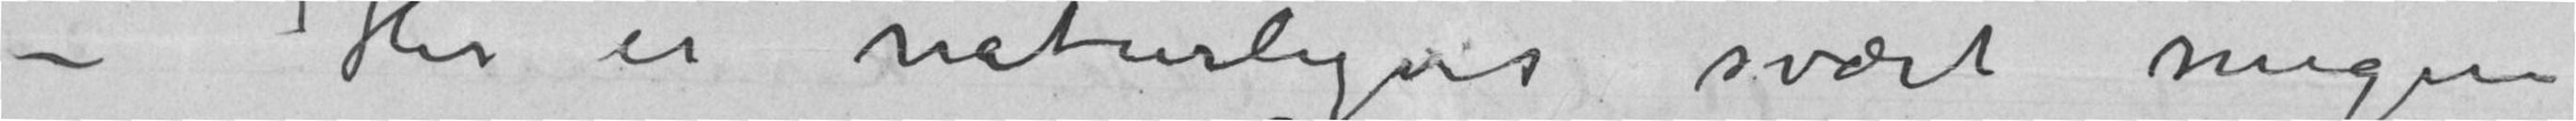– Her er naturligvis svært megen
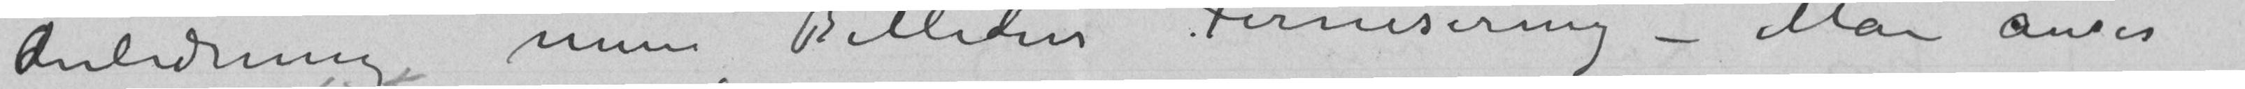Anledning mine Billeders Fernisering – Man anser
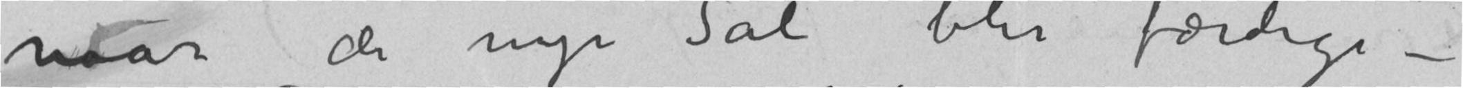naar de nye Sale blir færdige –
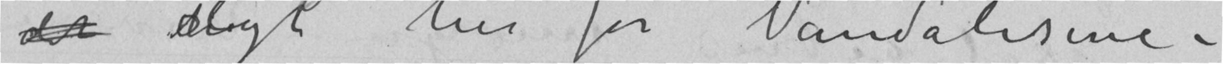det sligt her for Vandalisme –
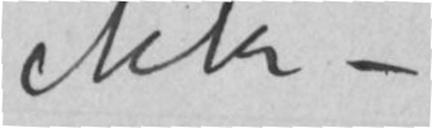ikke –
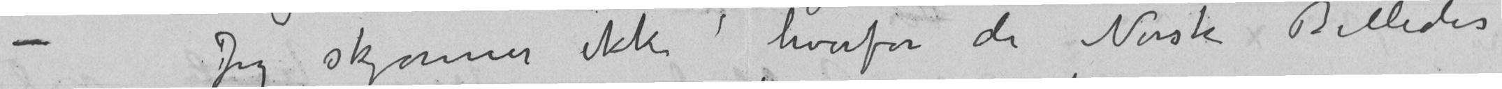– Jeg skjønner ikke hvorfor de Norske Billeder
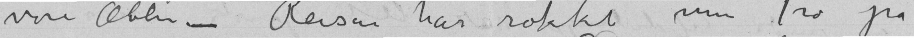vore Æbler – Reisen har rokket min Tro på
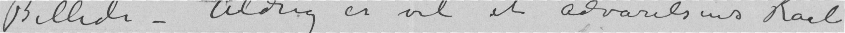Billede – Aldrig er vel et advarelsens Råd
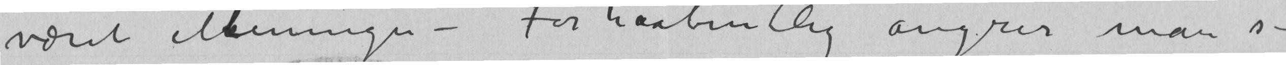været Meningen – Forhaabentlig angrer man sig
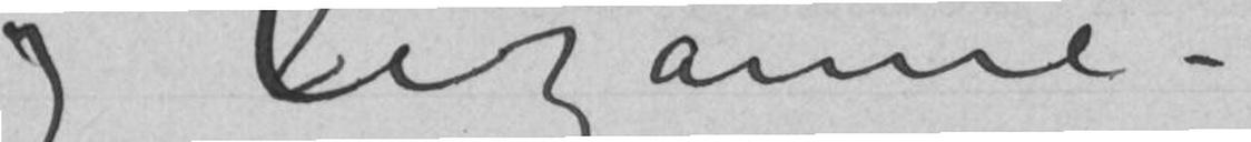og Cezanne –
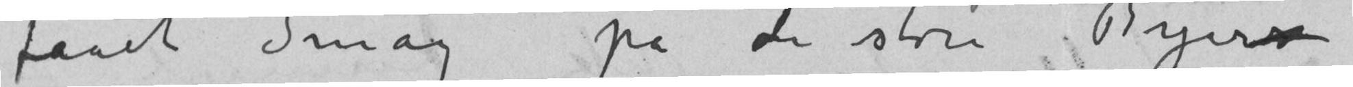faaet Smag på de store Byer
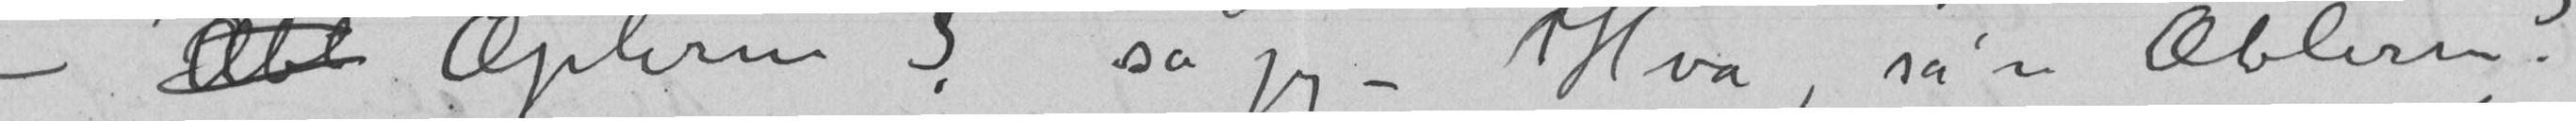– Æbl	Æplerne? sa jeg – Hva sa’n Æblerne?
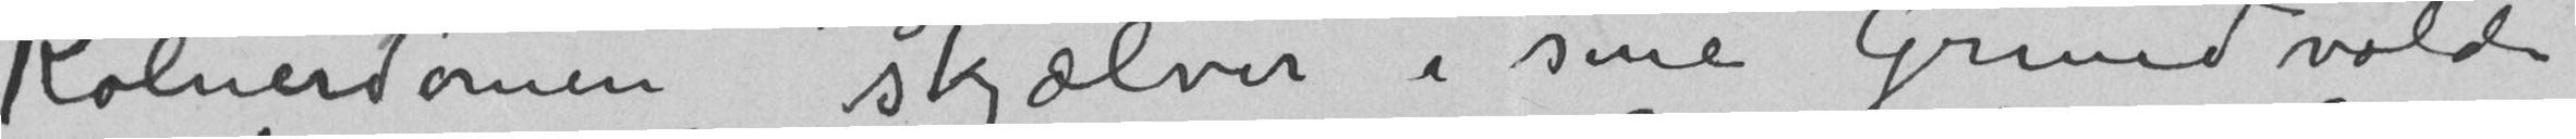Kølnerdomen skjælver i sine Grundvolde
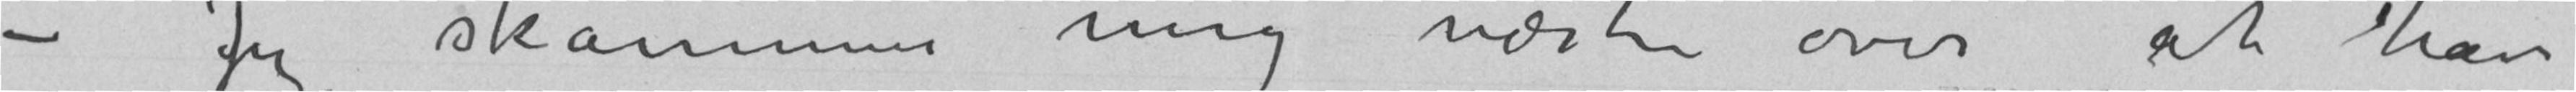– Jeg skammer mig nesten over at have
